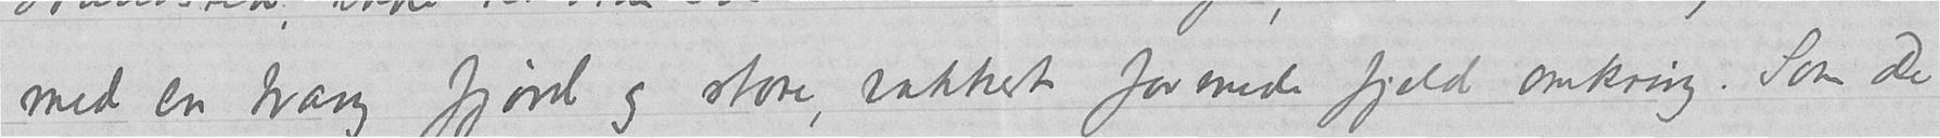med en trang fjord og store, vakkert formede fjeld omkring. Som De
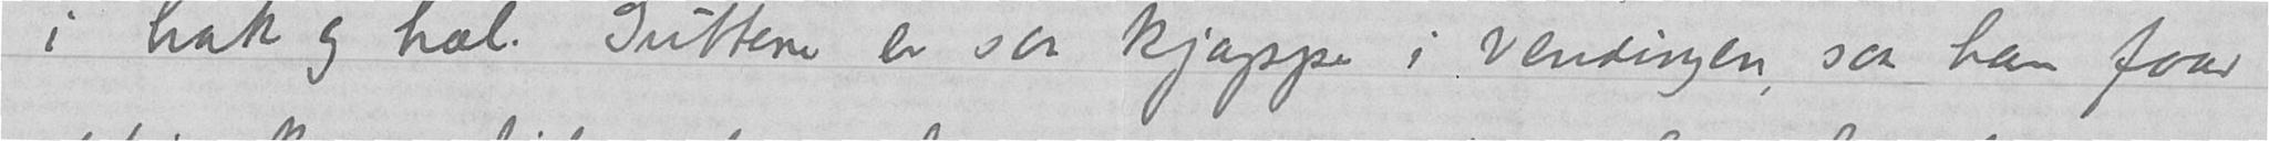i hak og hæl. Guttene er saa kjappe i vendingen, saa han faar
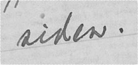siden.
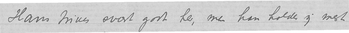Hans trives svært godt her, men han holder sig mest
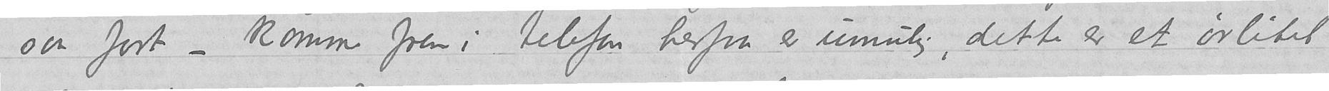saa fort – komme frem i telefon herfra er umulig, dette er et ørlitet
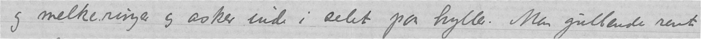og melkeringer og asker inde i selet paa hyller. Men gullende rent
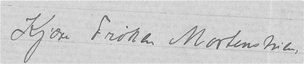Kjære Frøken Mortenstuen,
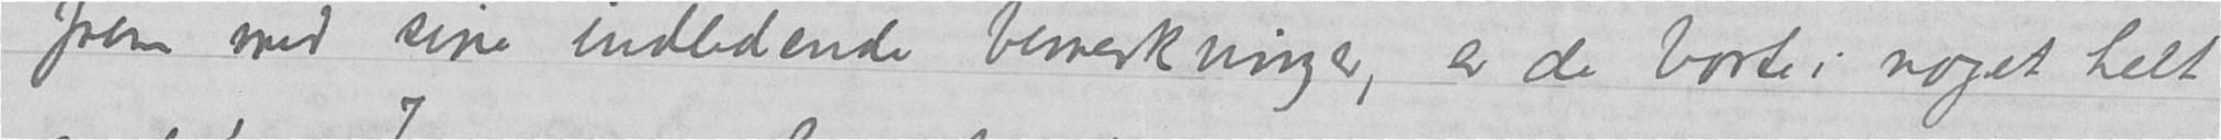frem med sine indledende bemerkninger, er de borte i noget helt
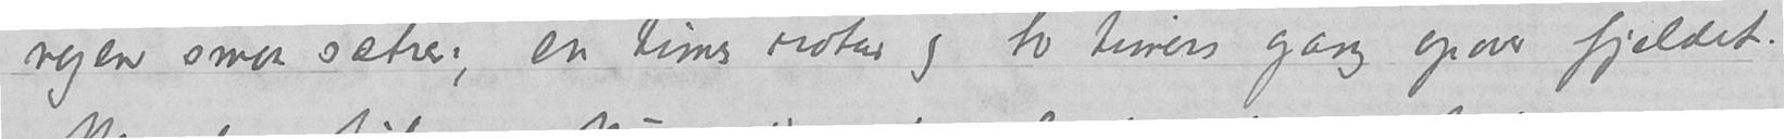nogen smaa sætrer; en tims rotur og to timers gang opover fjeldet.
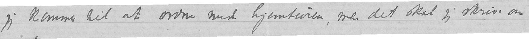jeg kommer til at ordne med hjemturen, men det skal jeg skrive om
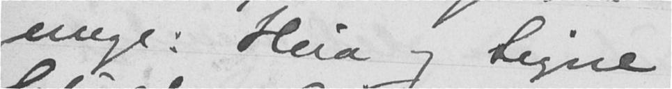unge: Heia og Signe
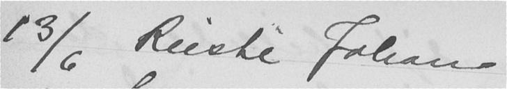13/6 Reiste Johan
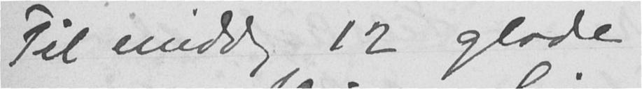Til middag 12 glade
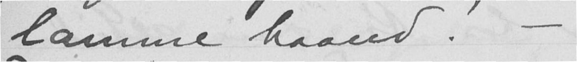lamme haand! -
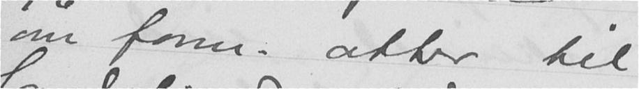om form. atter til
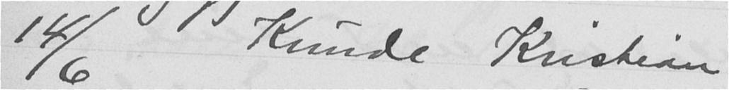14/6 Kunde Kristian
